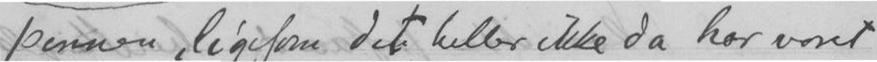pennen, ligesom det heller ikke da har været
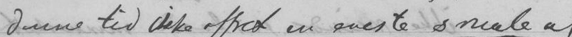denne tid ikke offret en eneste smule af
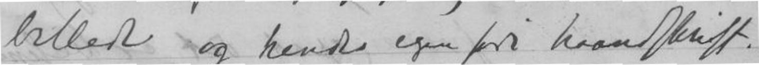billede og hendes egen fine haandskrift.
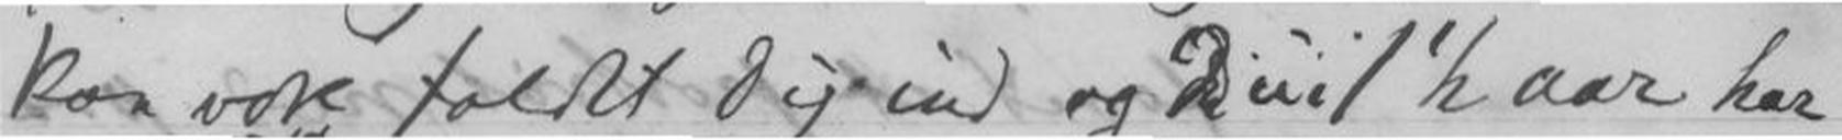kan være faldt dig ind og Du i 1½ aar har
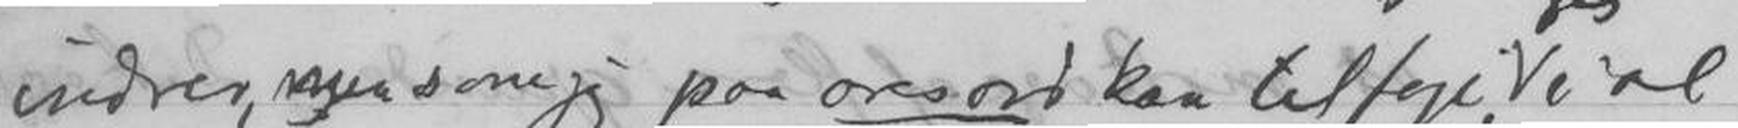indres, men som jeg paa æresord kan tilføje, i al
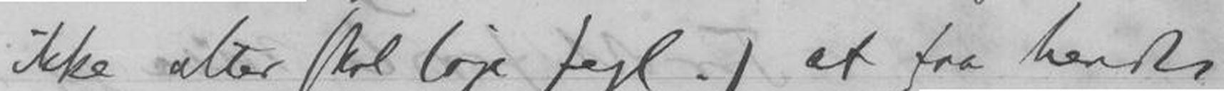ikke atter skal tage fejl.) at faa hendes
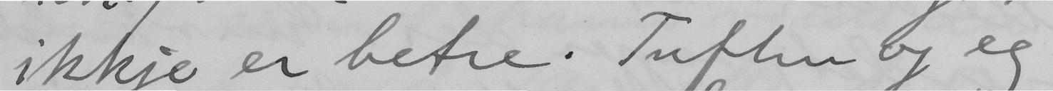ikkje er betre. Tuften og eg
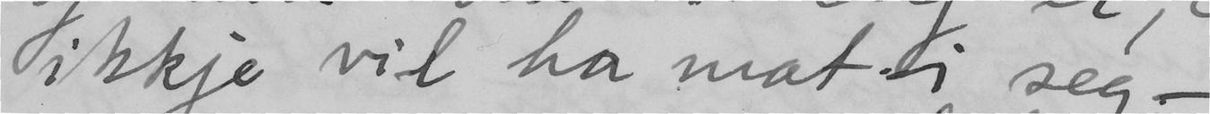ikkje vil ha mat i seg –
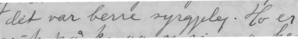det var berre syrgjeleg. Ho er
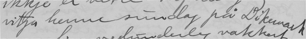vitja henne sundag på Dikemark
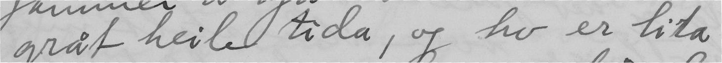gråt heile tida, og ho er lita
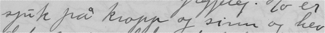sjuk på kropp og sinn og hev
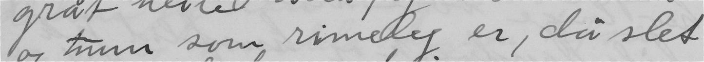og tunn som rimeleg er, då slet
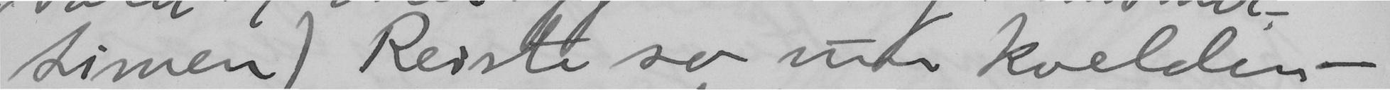timen) Reiste so um kvelden –
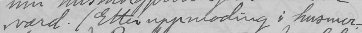værd. (Etteruppmoding i husmor-
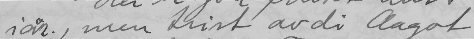iår, men trist avdi Aagot
